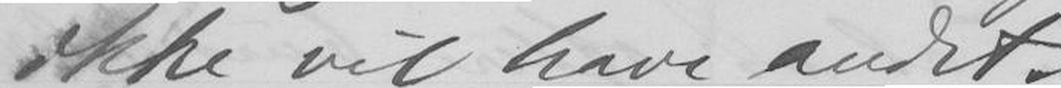ikke vil have andet.
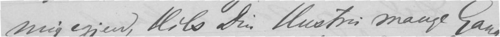mig igjen, Hils Din Hustru mange Gange
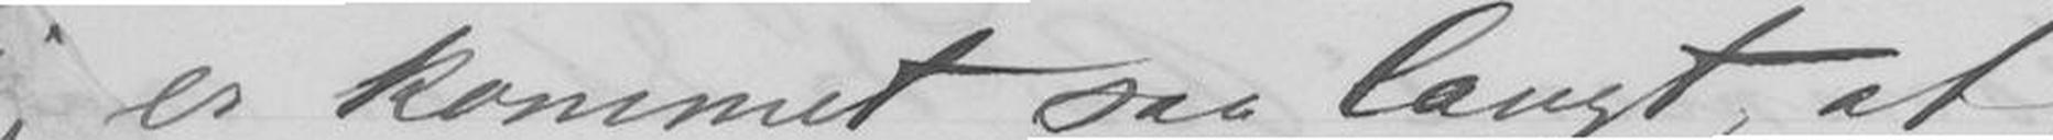jeg er kommet saa langt, at
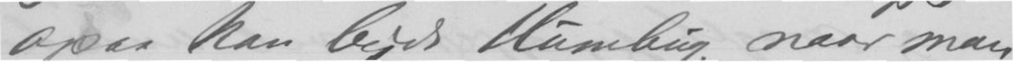ogsaa kan byde Humbug, naar man
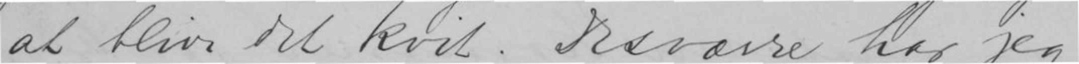at blive det kvit. Desværre har jeg
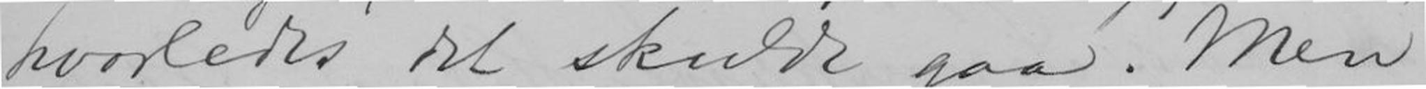hvorledes det skulde gaa. Men
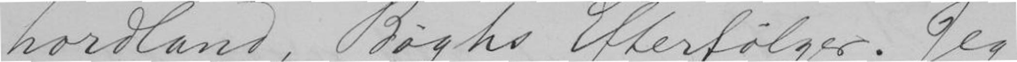hordland, Bøghs Efterfølger. Jeg
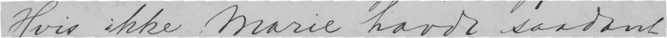Hvis ikke Marie havde saadant
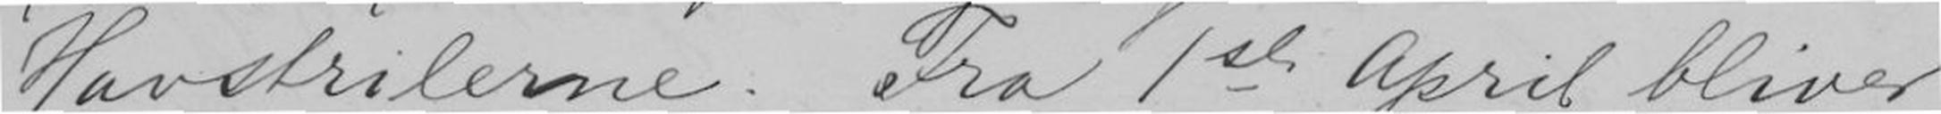Havstrilerne. Fra 1ste April bliver
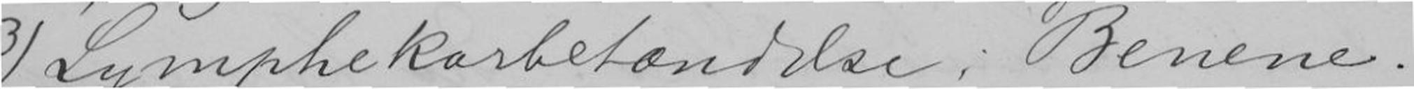3) Lymphekarbetændelse i Benene.
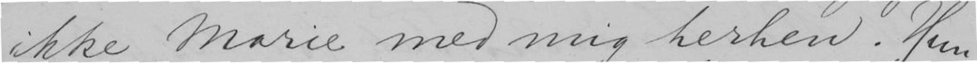ikke Marie med mig herhen. Hun
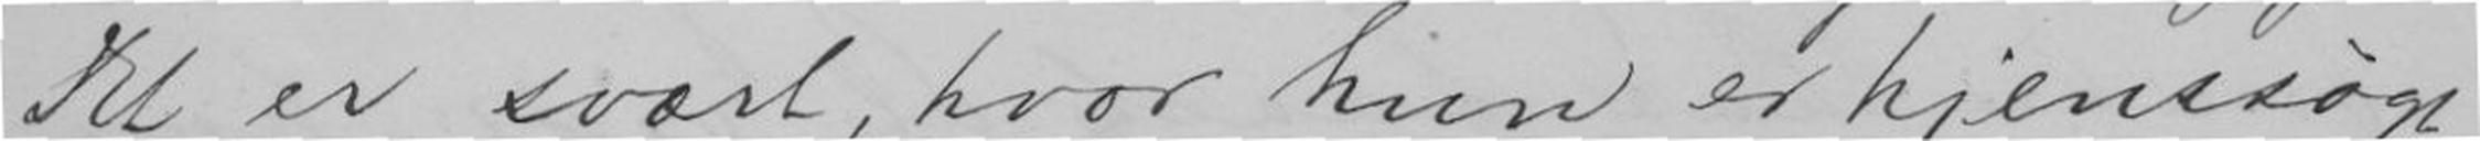Det er svært, hvor hun er hjemsøgt
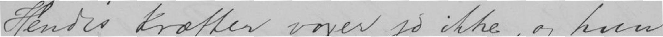Hendes Kræfter voxer jo ikke, og hun
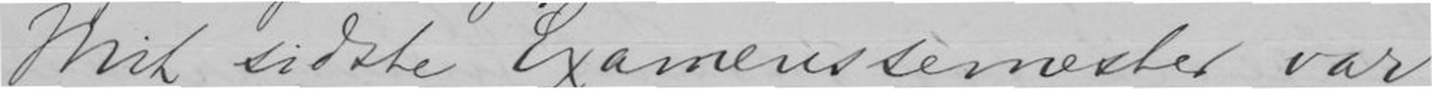Mit sidste Examenssemester var
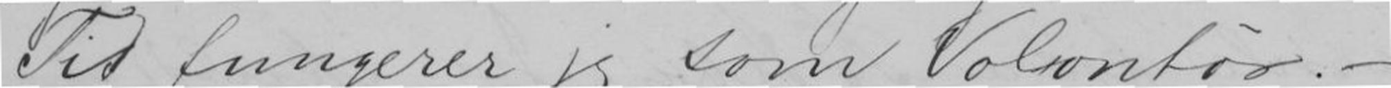Tid fungerer jeg som Volontør.-
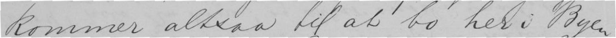kommer altsaa til at bo her i Byen
